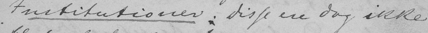Institutioner: disse ere dog ikke
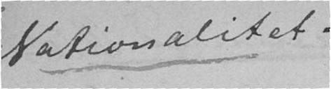Nationalitet –
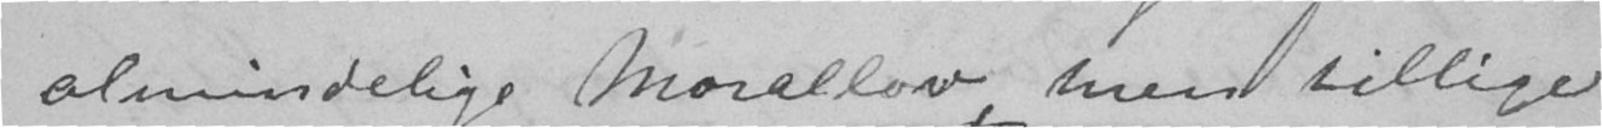almindelige Morallov, men tillige
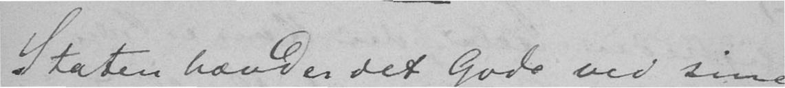Staten hævder det Gode ved sine
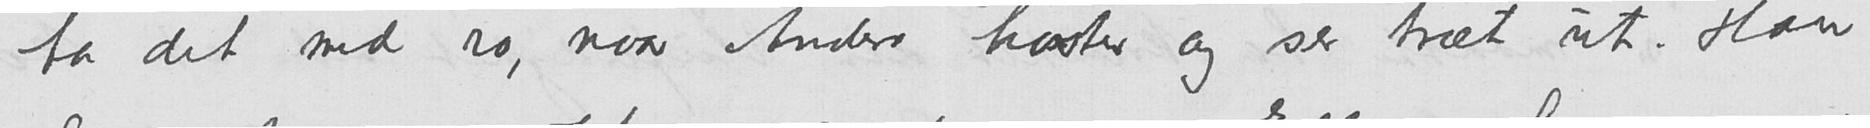ta det med ro, naar Anders hoster og ser træt ut. Han
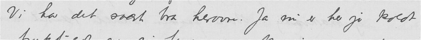Vi har det svært bra herovre. Ja nu er her jo koldt
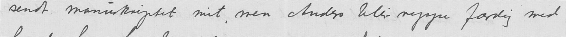sendt manuskriptet mit, men Anders blir neppe færdig med
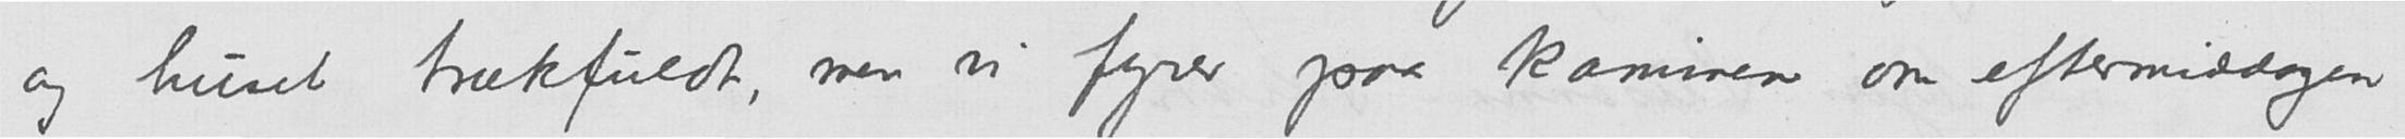og huset trækfuldt, men vi fyrer paa kaminen om eftermiddagen
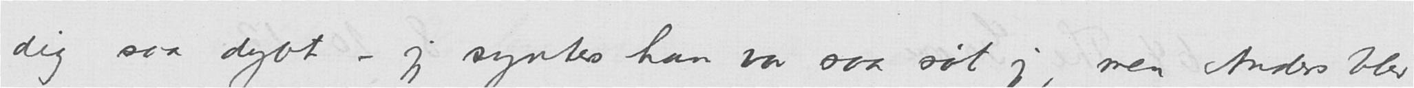dig saa dybt – jeg syntes han var saa søt jeg, men Anders blev
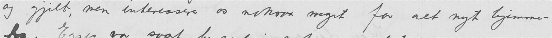og gjilt, men interesserer os noksaa meget for alt nyt hjemme-
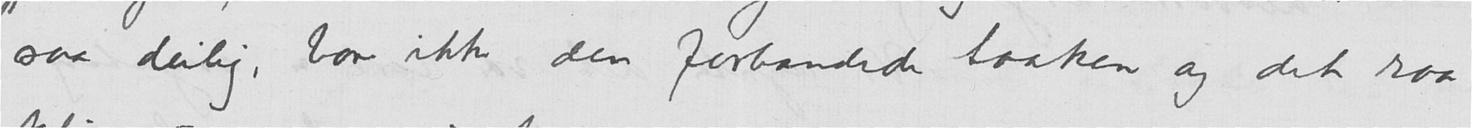saa deilig, bare ikke den forbandede taaken og det raa
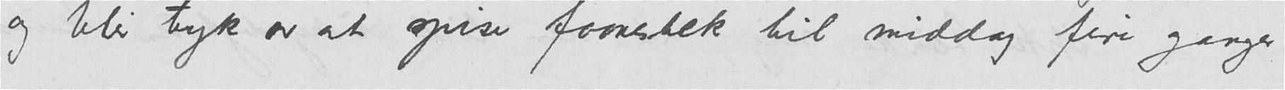og blir tyk av at spise faarestek til middag fire ganger
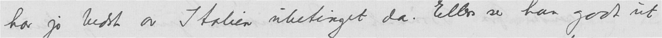har jo bedst av Italien ubetinget da. Ellers ser han godt ut
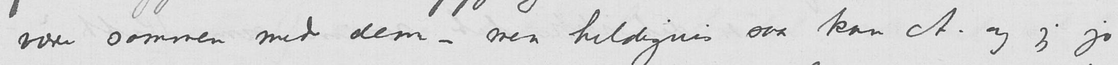være sammen med dem – men heldigvis saa kan A. og jeg jo
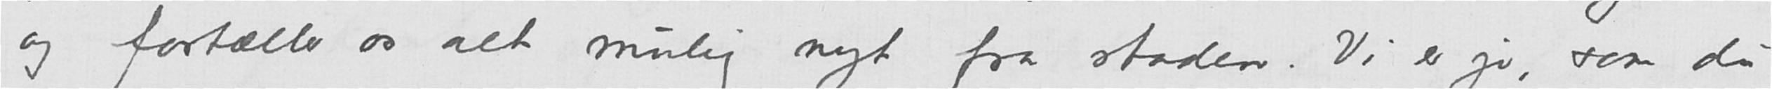og fortæller os alt mulig nyt fra staden. Vi er jo, som du
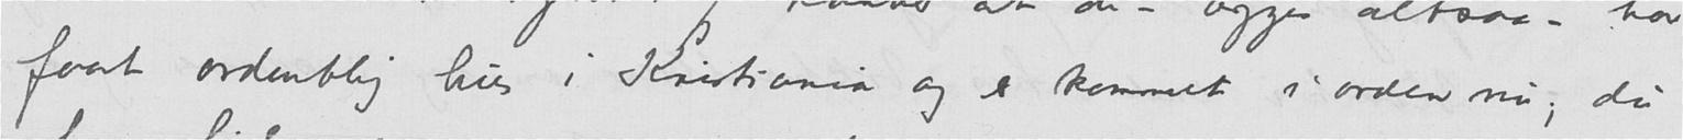faat ordentlig hus i Kristiania og er kommet i orden nu; du
